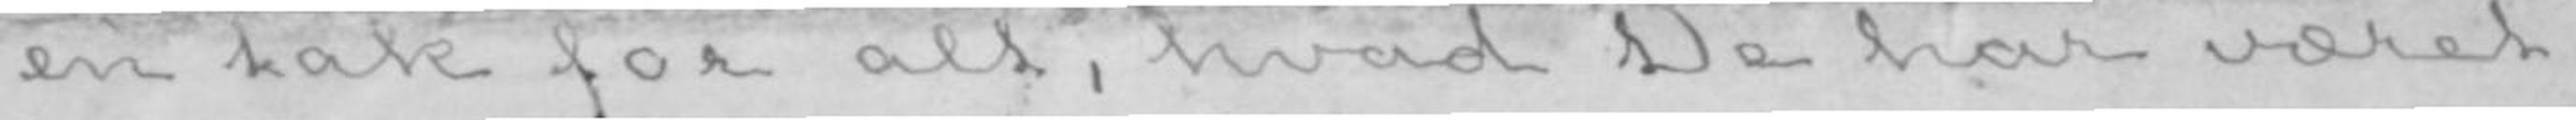en tak for alt, hvad De har været
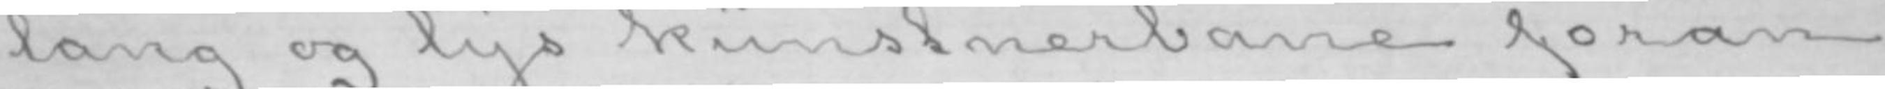lang og lys kunstnerbane foran
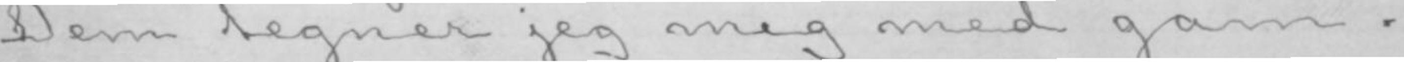Dem tegner jeg mig med gam-
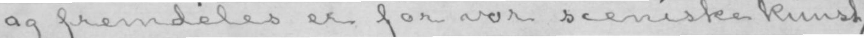og fremdeles er for vor sceniske kunst,
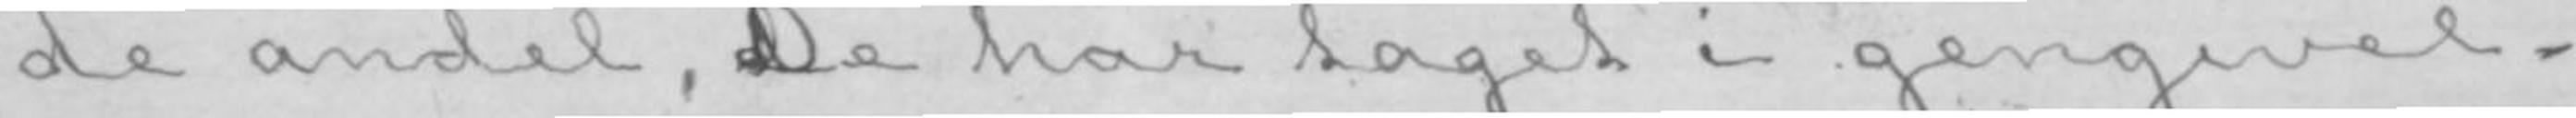de andel, dDe har taget i gengivel-
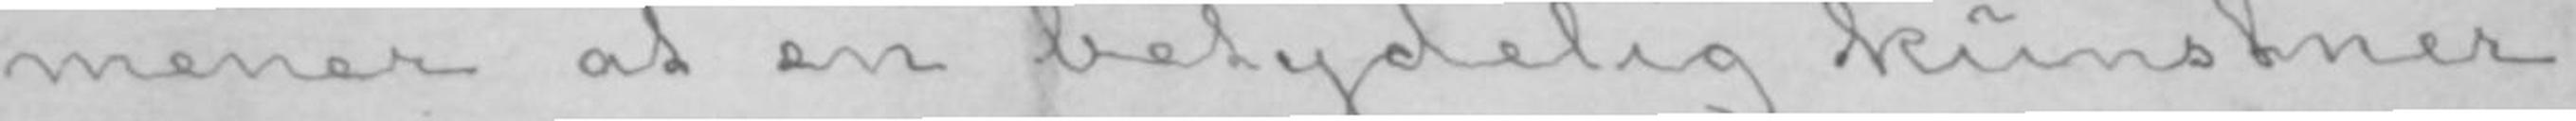mener at en betydelig kunstner,
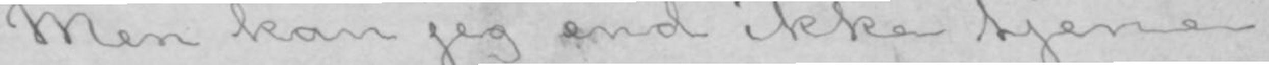Men kan jeg end ikke tjene
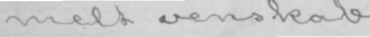melt venskab
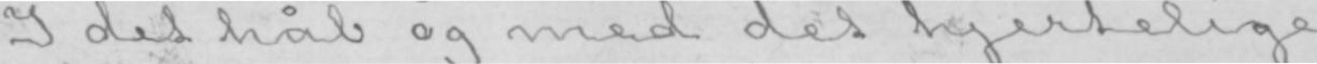I det håb og med det hjertelige
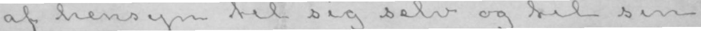af hensyn til sig selv og til sin
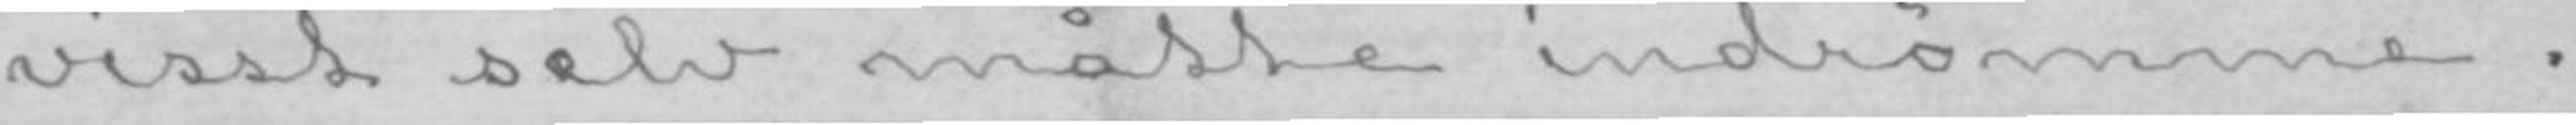visst selv måtte indrømme.
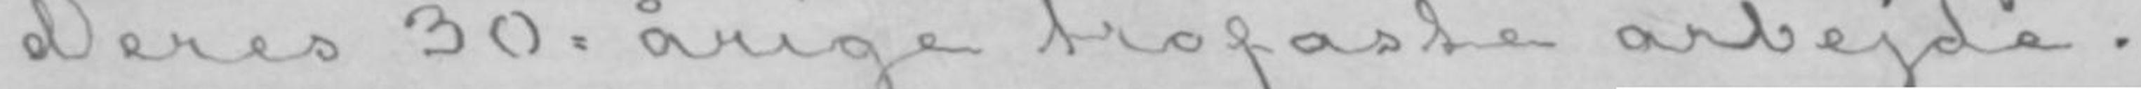Deres 30-årige trofaste arbejde.
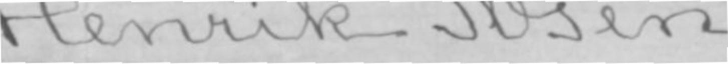Henrik Ibsen.
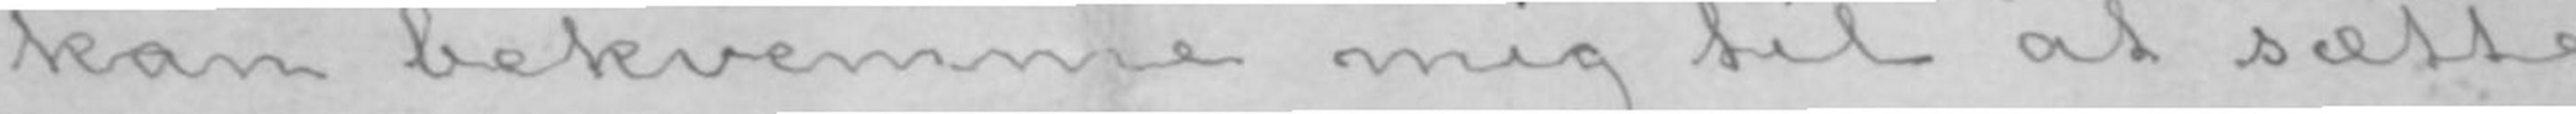kan bekvemme mig til at sætte
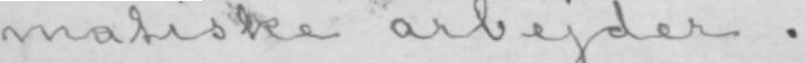matiske arbejder.
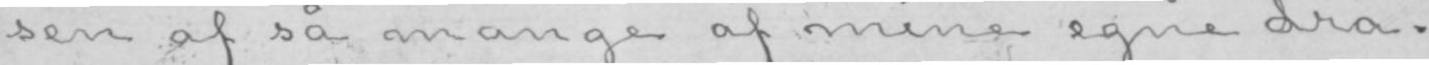sen af så mange af mine egne dra-
